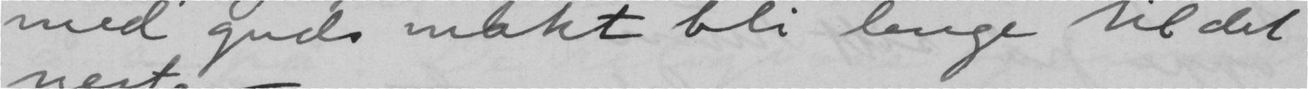med guds makt bli lenge til det
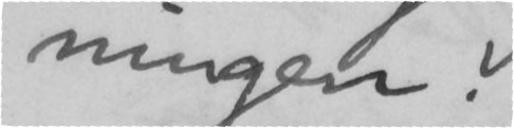ningen!
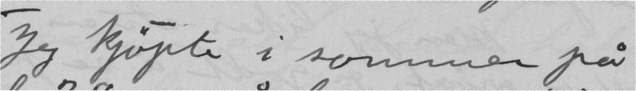Jeg kjøpte i sommer på
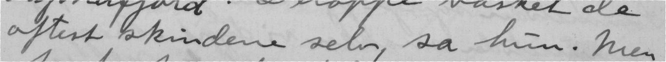oftest skindene selv sa hun. Men
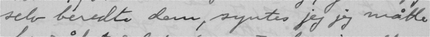selv beredte dem, syntes jeg jeg måtte
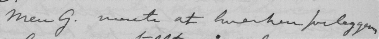Men G. mente at hverken forleggeren
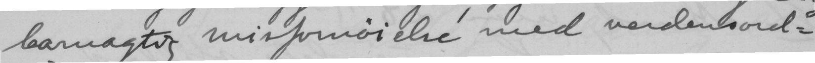barnagtig misfornøielse med verdensord-
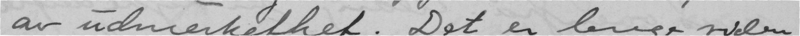av udmerkethet. Det er lenge siden
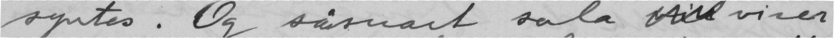syntes. Og såsnart sola  viser
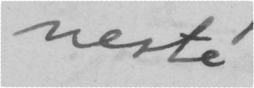neste
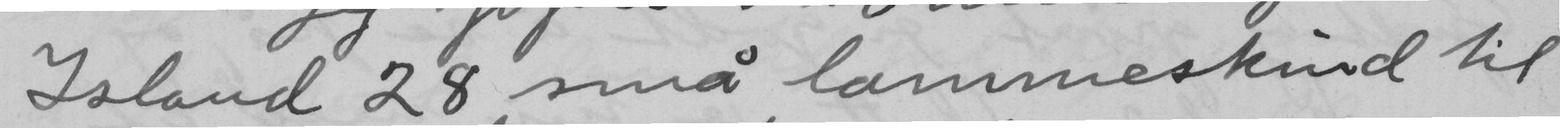Island 28 små lammeskind til
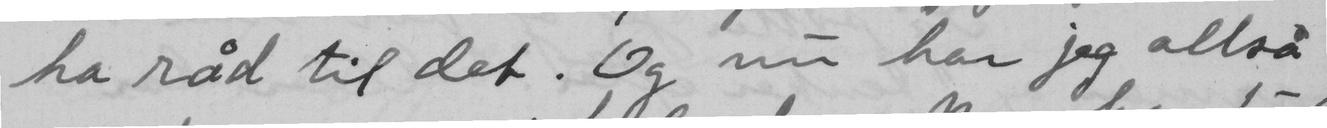ha råd til det. Og nu har jeg altså
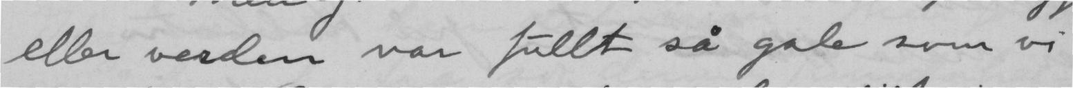eller verden var fullt så gale som vi
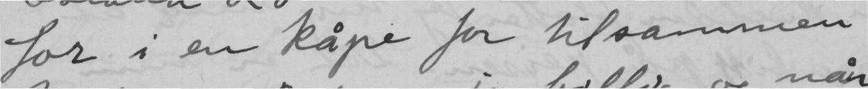for i en kåpe for tilsammen
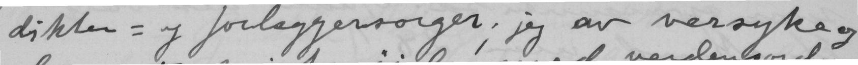dikter - og forleggersorger, jeg av versyke og
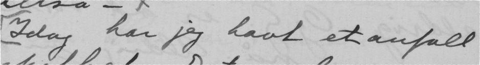Idag har jeg havt et anfall
